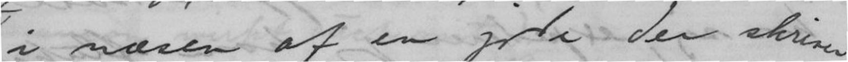i næsen af en jöde der skriver
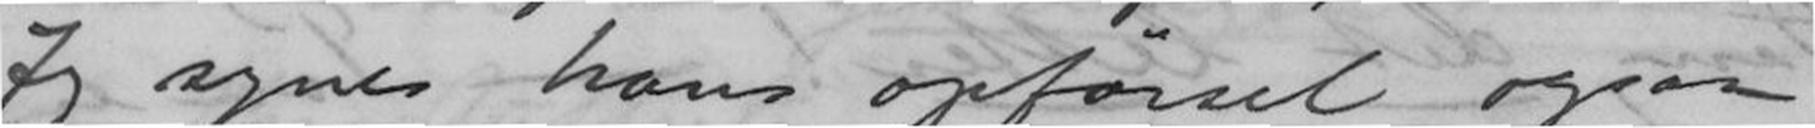Jeg synes hans opförsel ogsaa
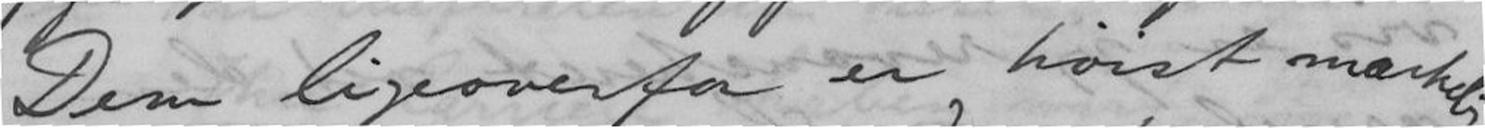Dem ligeoverfor er höist mærkelig
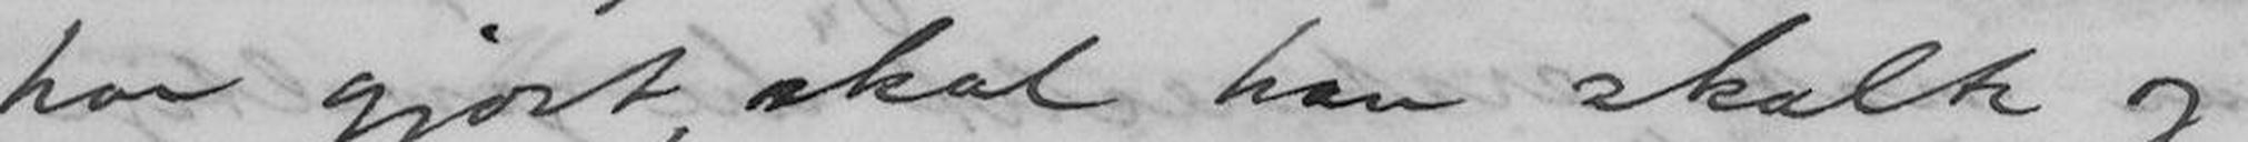har gjort, skal han skalte og
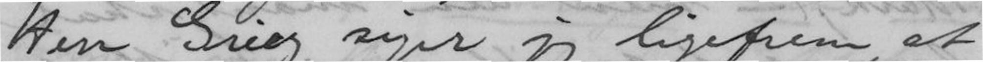Herr Grieg siger jeg ligefrem, at
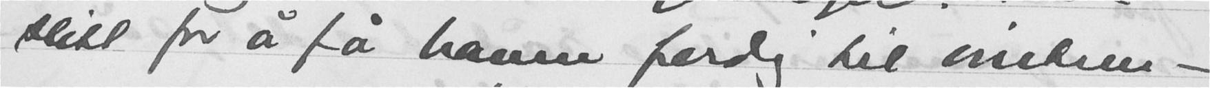slitt for å få haven ferdig til vinteren -
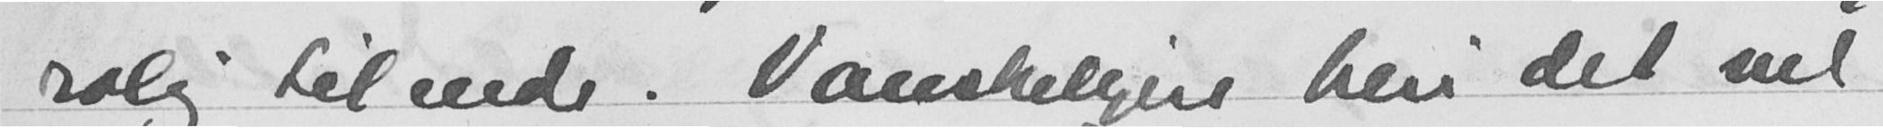rolig til ende. Vanskeligere blir det vel
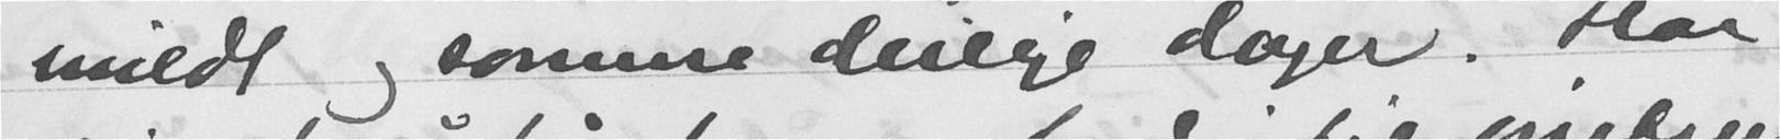mildt og somme deilige dager. Har
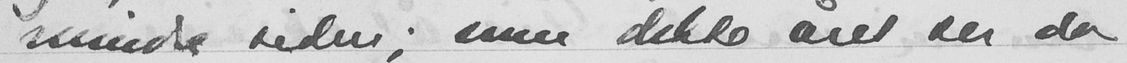mindre siden; men dette året ser da
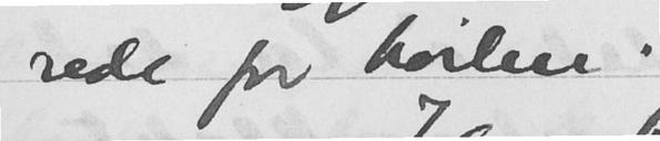rede for høslen.
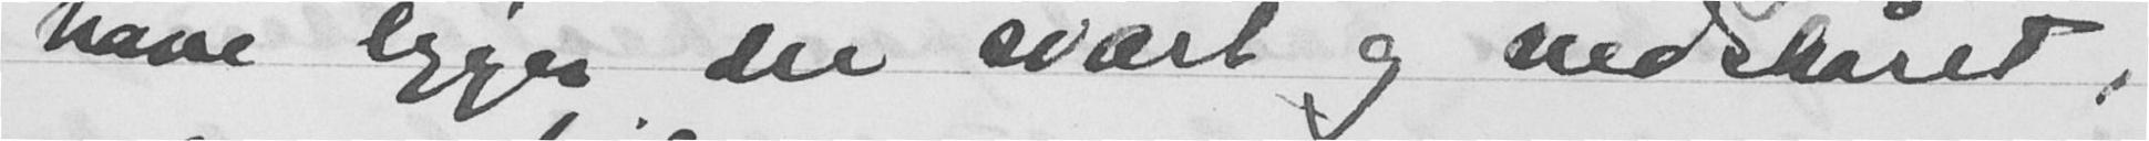have ligges der svært og nedskåret,
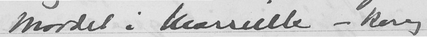Mordet i Marseille - kong
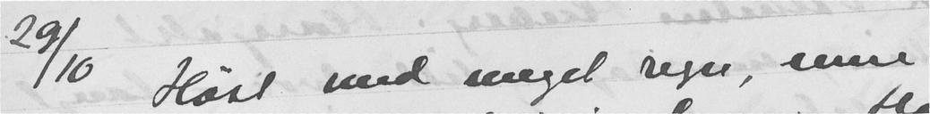29/10 Høst med meget regn, men
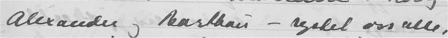Alexander og Barthan - rystet oss alle,
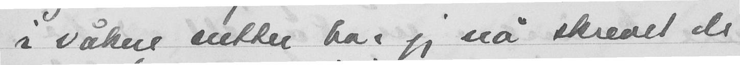i våkne netter har jeg nå skrevet de
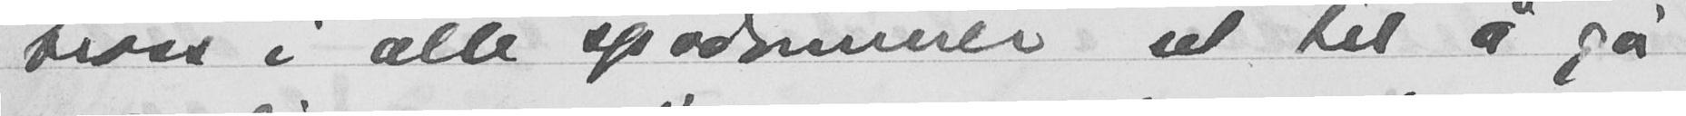tross i alle spådommer ut til å gå
